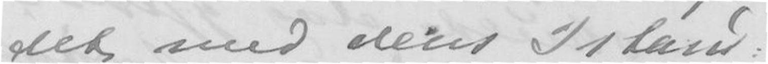det med deres Istand-
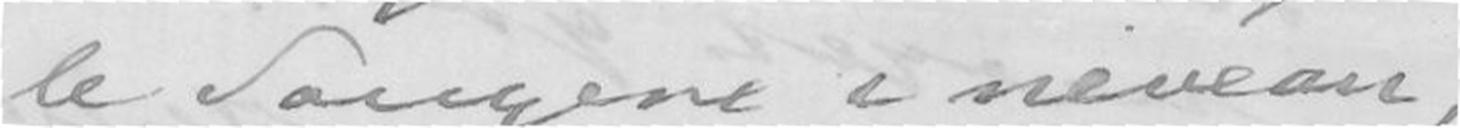le Sangene i niveau,
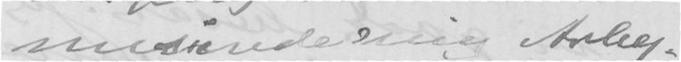misunde mig Arbej-
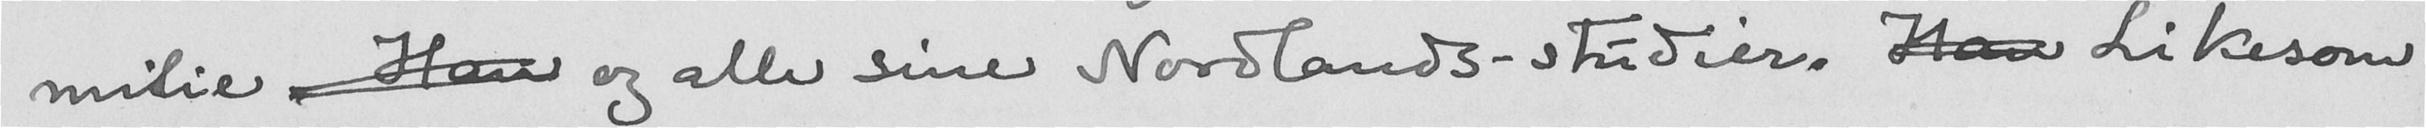milie Han og alle sine Nordlands-studier. Han Likesom
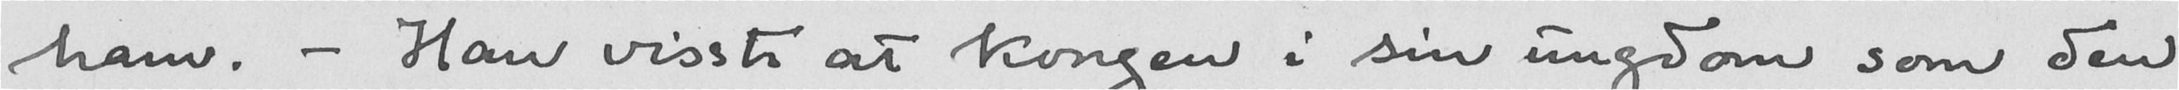ham. - Han visste at kongen i sin ungdom som den
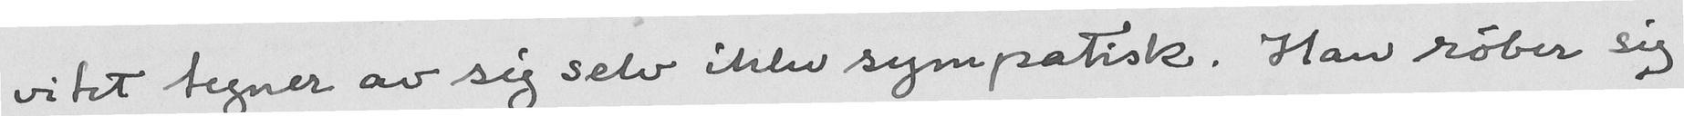vitet tegner av sig selv ikke sympatisk. Han røber sig
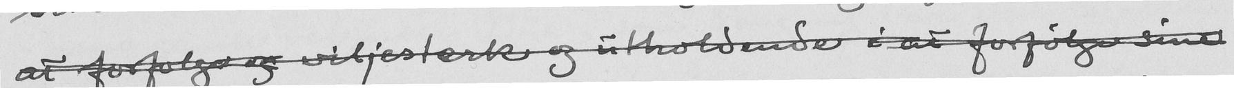at forfølge og viljestærk og utholdende i at forfølge sine
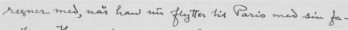regner med, når han nu flytter til Paris med sin fa-
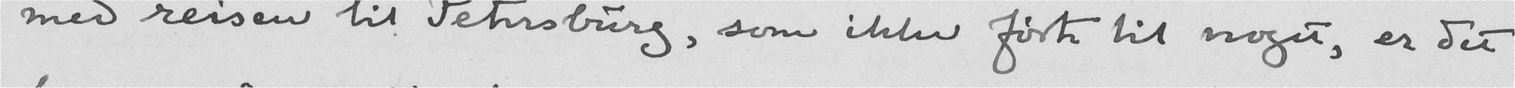med reisen til Petersburg, som ikke førte til noget, er det
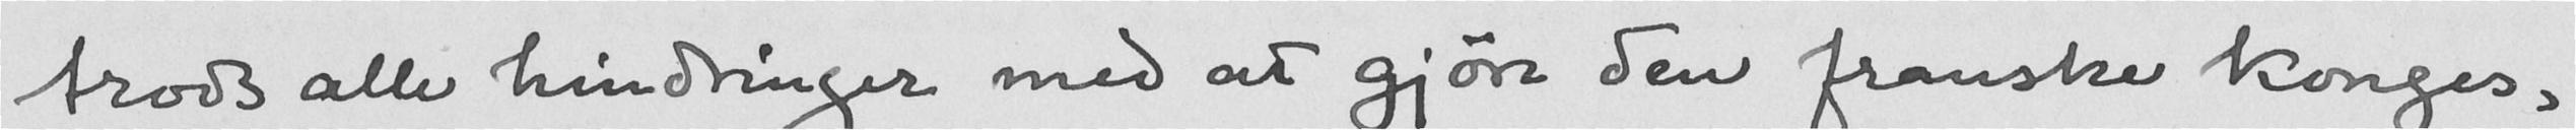trods alle hindringer med at gjøre den franske konges,
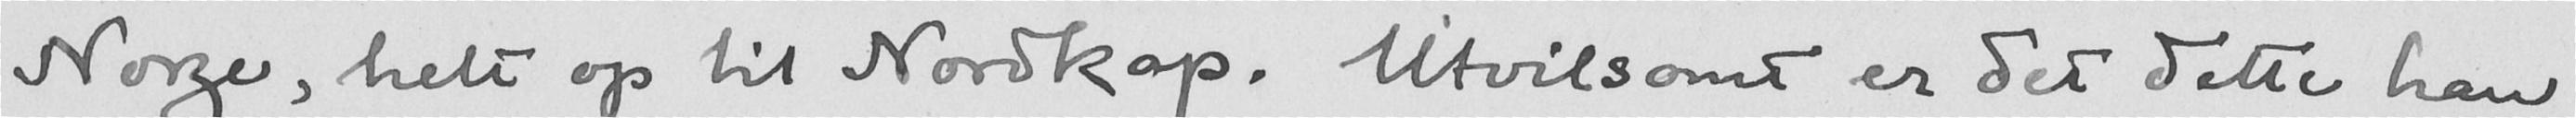Norge, helt op til Nordkap. Utvilsomt er det dette han
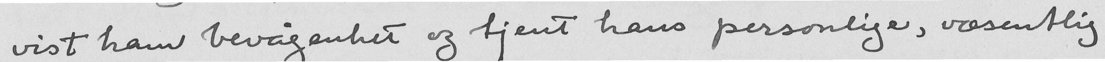vist ham bevågenhet og tjent hans personlige, væsentlig
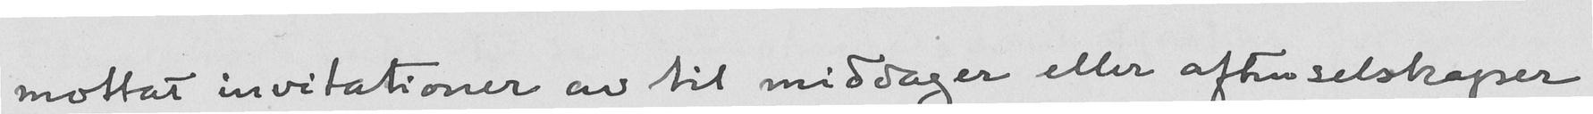mottat invitationer av til middager eller aftenselskaper
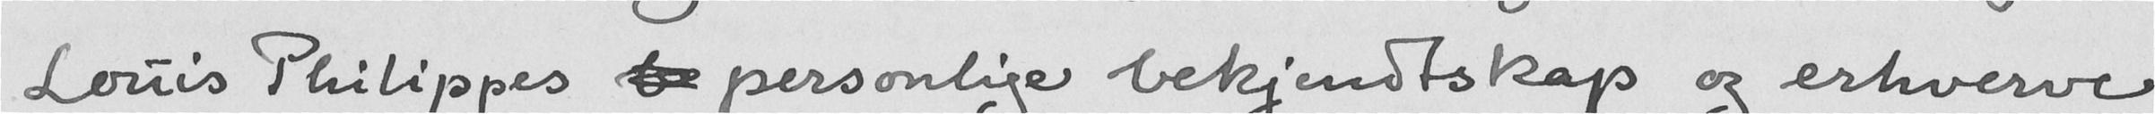Louis Philippes b personlige bekjendtskap og erhverve
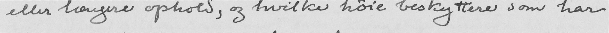eller længere ophold, og hvilke høie beskyttere som har
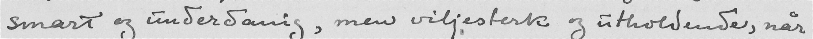smart og underdanig, men viljesterk og utholdende, når
